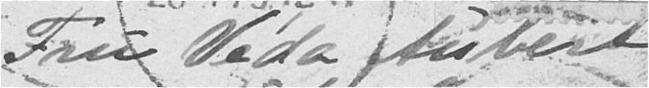Frue Veda Aubert
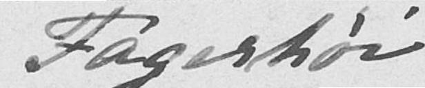Fagerhøi
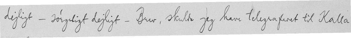dejligt - sørgeligt dejligt - Brev, skulde jeg have telegraferet til Kalla
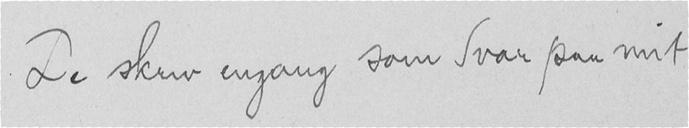De skrev engang som Svar paa mit
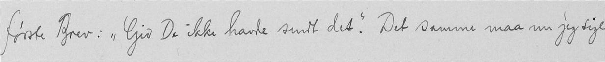første Brev: "Gid De ikke havde sendt det". Det samme maa nu jeg sige
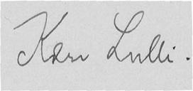Kære Lulli.
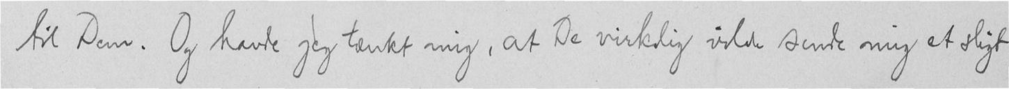til Dem. Og havde jeg tænkt mig, at De virkelig vilde sende mig et sligt
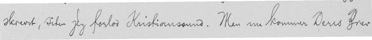skrevet, siden jeg forlod Kristianssund. Men nu kommer Deres Brev
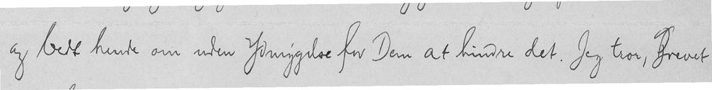og bedt hende om uden Ydmygelse for Dem at hindre det. Jeg tror, Brevet
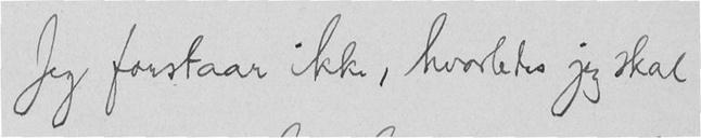Jeg forstaar ikke, hvorledes jeg skal
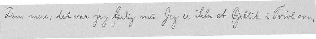Dem mere, det var jeg færdig med. Jeg er ikke et Øjeblik i Tvivl om,
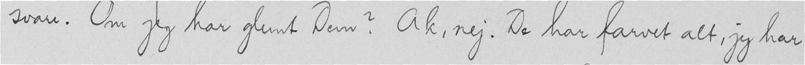svare. Om jeg har glemt Dem? Ak, nej. De har farvet alt, jeg har
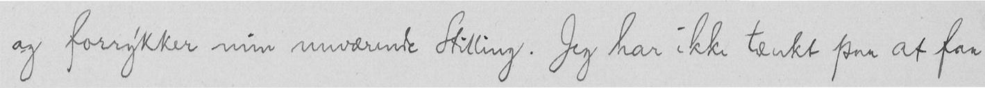og forrykker min nuværende Stilling. Jeg har ikke tænkt paa at faa
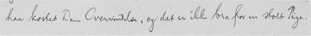har kostet Dem Overvindelse, og det er ikke bra for en stolt Pige.
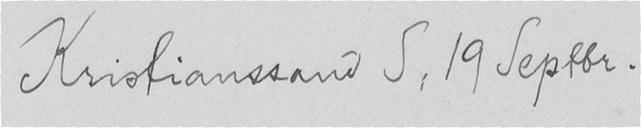Kristianssand S, 19 Septbr.
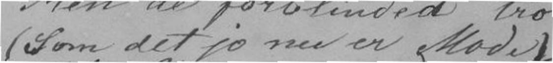(Som det jo nu er Mode)
